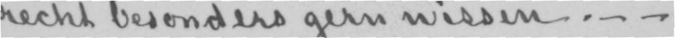recht besonders gern wissen.--
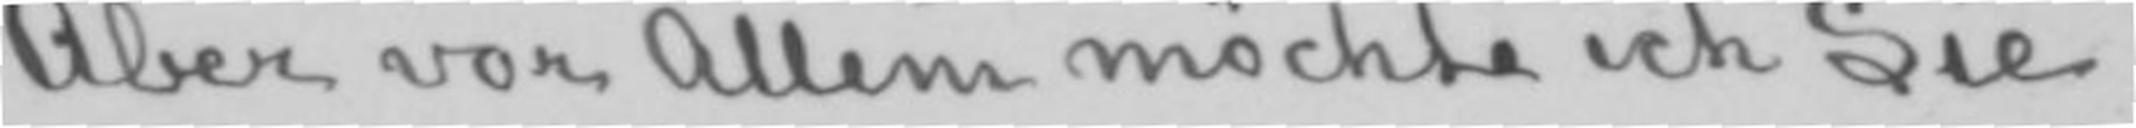Aber vor Allem möchte ich Sie
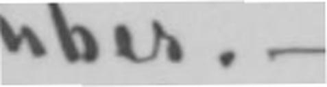über.--
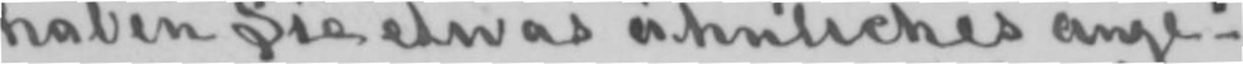haben Sie etwas ähnliches ange-
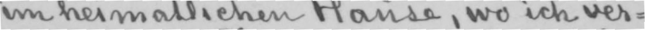im heimatlichen Hause, wo ich ver-
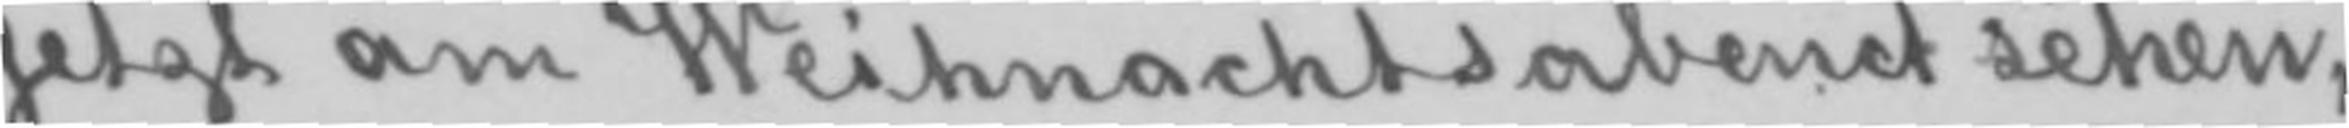jetzt am Weihnachtsabend sehen,
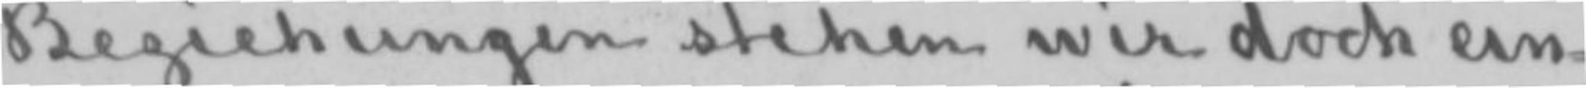Beziehungen stehen wir doch ein-
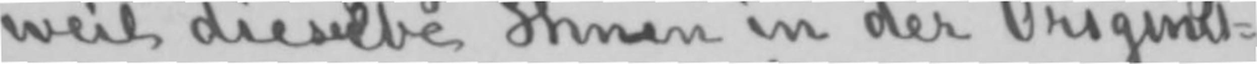weil dieselbe Ihnen in der Original-
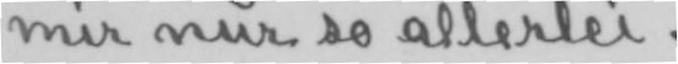mir nur so allerlei.
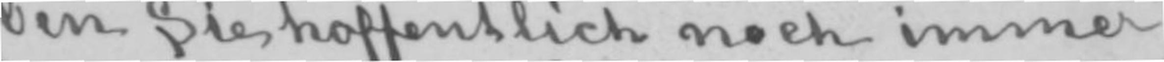ben Sie hoffentlich noch immer
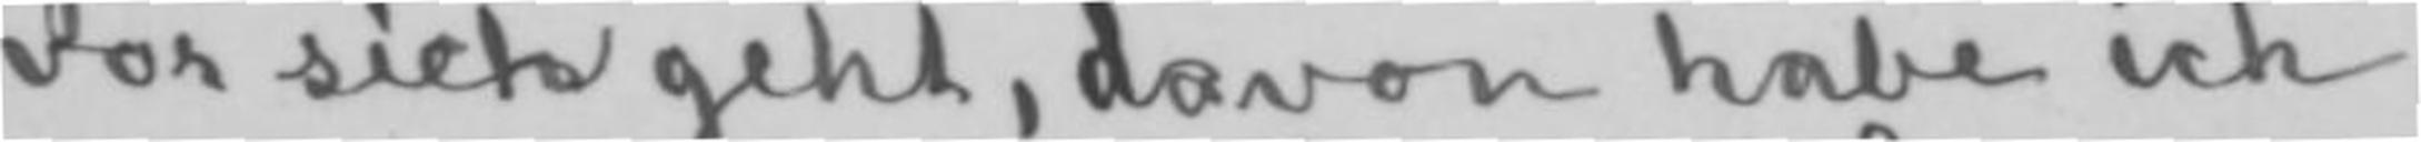vor sich geht, davon habe ich
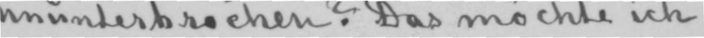ununterbrochen? Das möchte ich
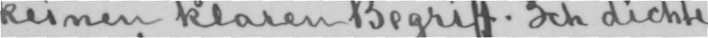keinen klaren Begriff. Ich dichte
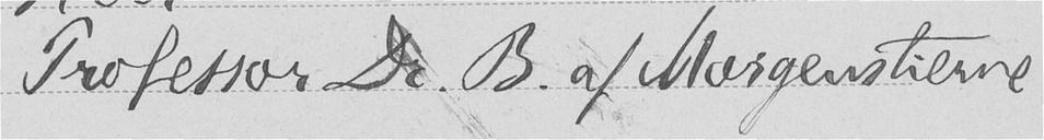Professor Dr. B. af Morgenstierne
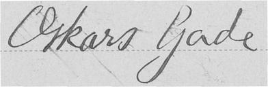Oskars Gade.
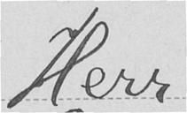Herr
